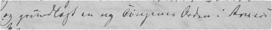og grundlagt en ny Tingenes Orden i Ameri-
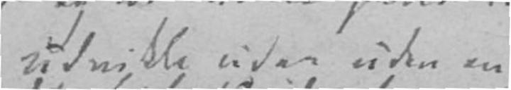udvikle uden uden en
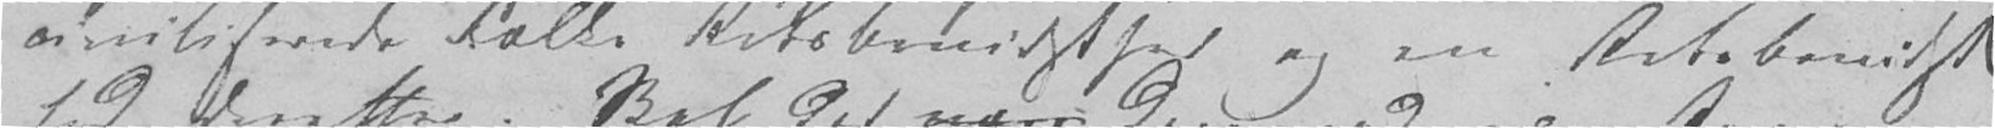civiliserede Folks Retsbevidsthed og en Retsbevidst-
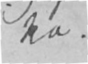ka.
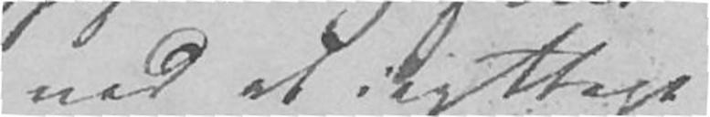ved at iagttage
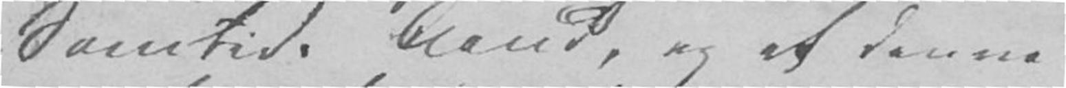Samtids Aand, og at denne
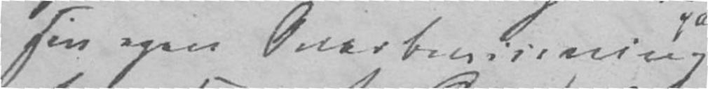sin egen Overbeviisning
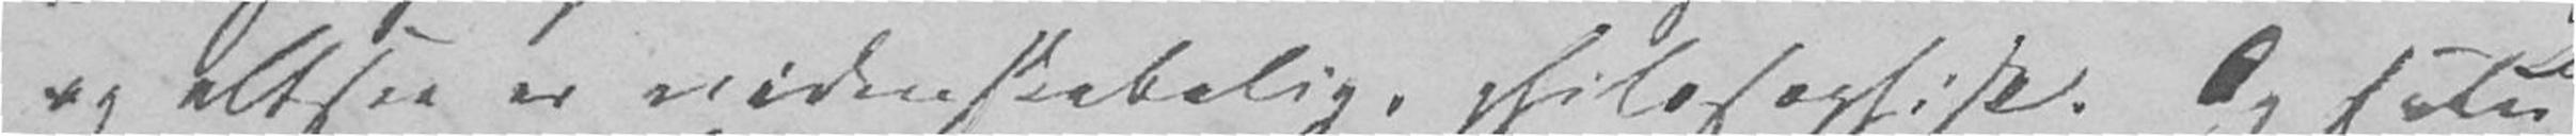og altsaa er videnskabelig, philosophisk. Og selv
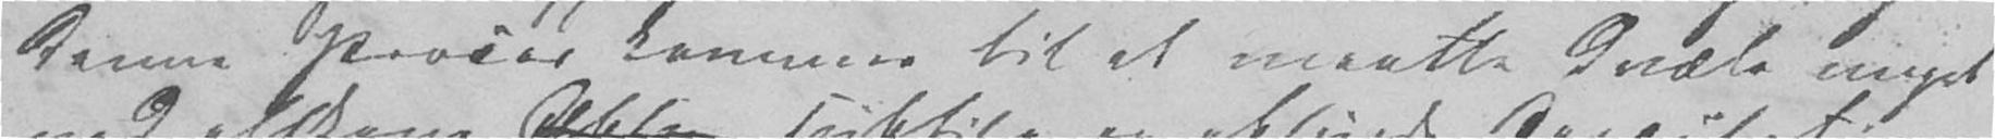denne Proces kommer til at maatte dvæle meget
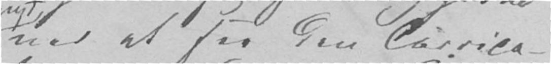ved at see den Carrica-
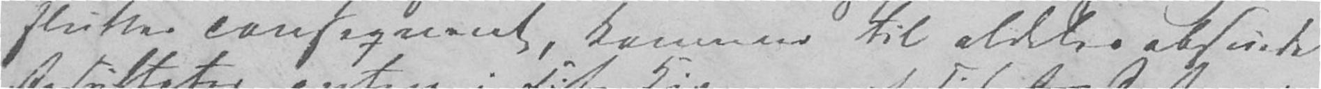slutter conseqvent, kommer til aldeles absurde
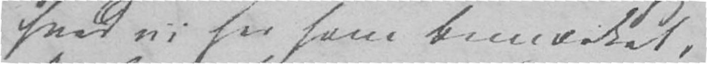hvad vi her have bemærket,
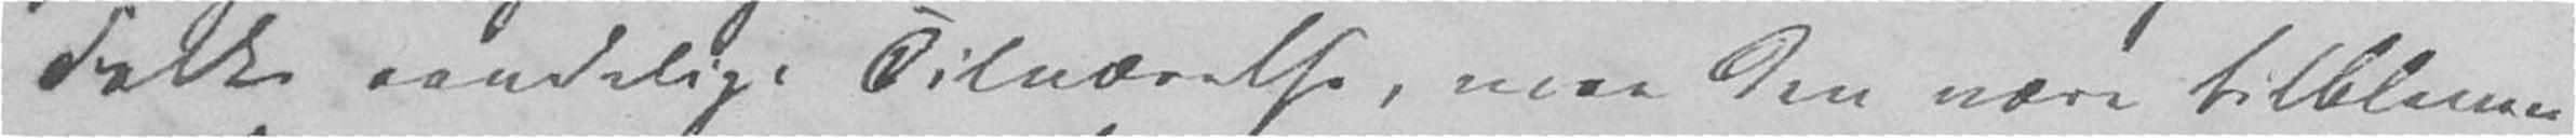Folks aandelige Tilværelse, maa den være tilbleven
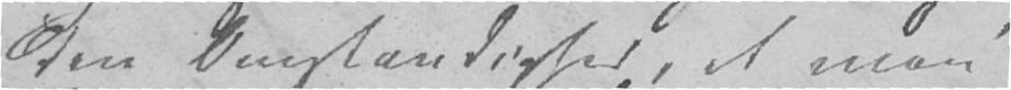den Omstændighed, at man
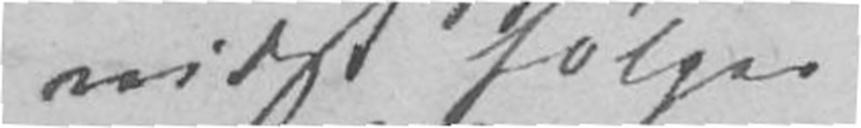vidst følger
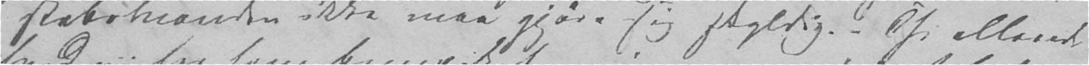skabsmanden ikke maa gjøre sig skyldig. – Thi allerede
